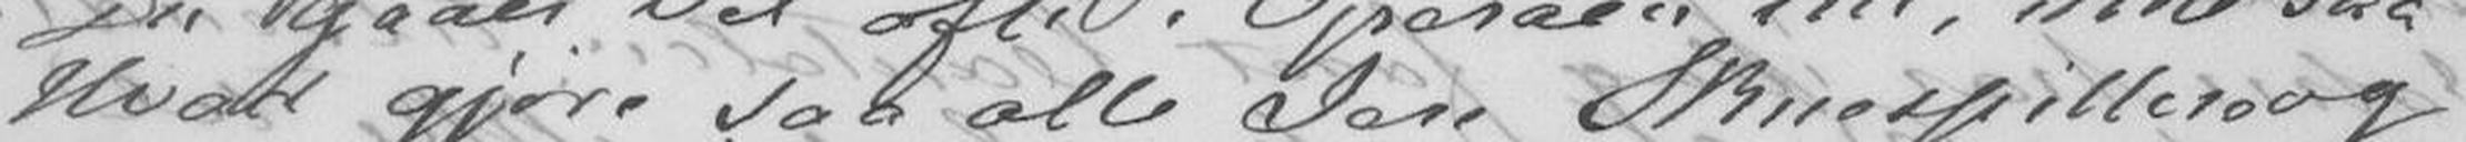Hvad gjøre saa alle Jere Skuespillere og
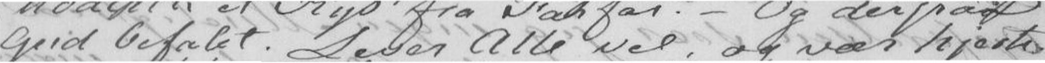Gud befalet. Lever Alle vel, og vær hjerte-
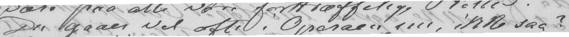Du gaaer vel ofte i Operaen nu, ikke saa?
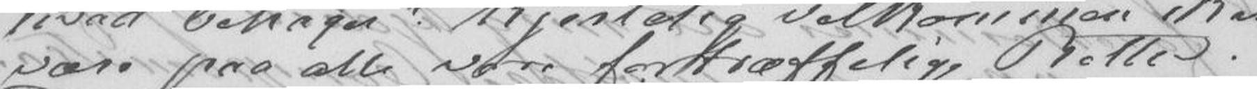være paa alle vore fortræffelige Rette.
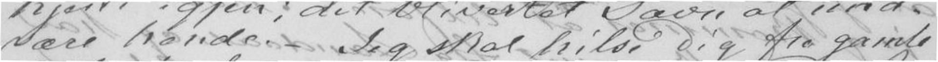være hende. - Jeg skal hilse Dig fra gamle
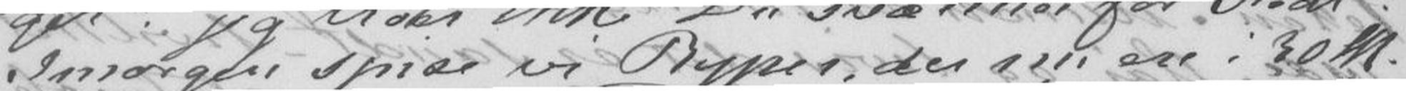Imorgen spise vi Ryper, der nu ere i 30 Sk.
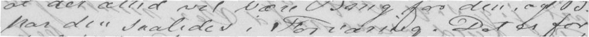har den saaledes i Forvaring. Det er for
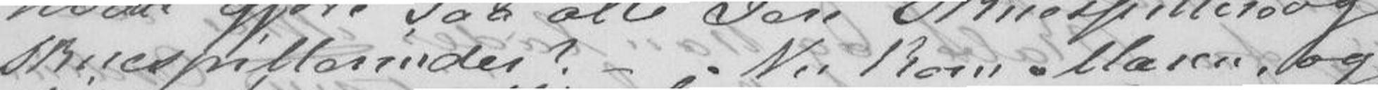Skuespillerinder? - Nu kom Maren, og
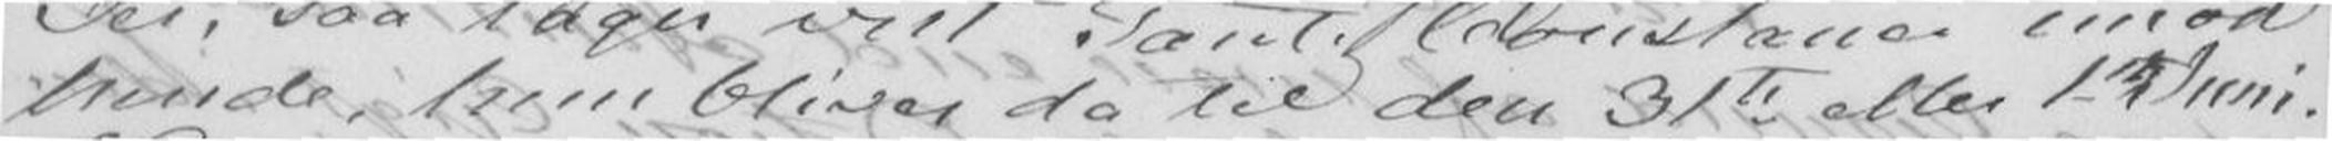hende, hun bliver da til den 31te eller 1ste Juni.
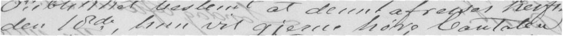den 18de, hun vil gjerne høre Cantaten
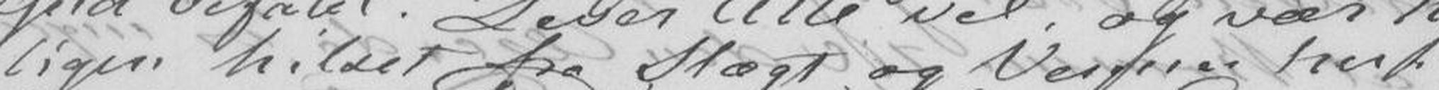ligen hilset fra Slægt og Venner her.
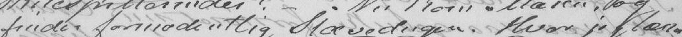finder formodentlig Slævedugen. Hvor jeg læn-
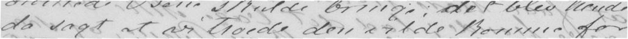da sagt at vi troede den vilde komme for
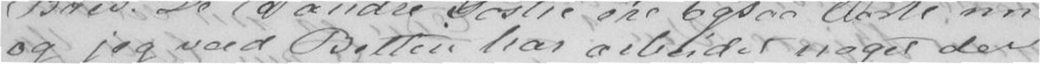og jeg veed Betten har arbeidet noget der
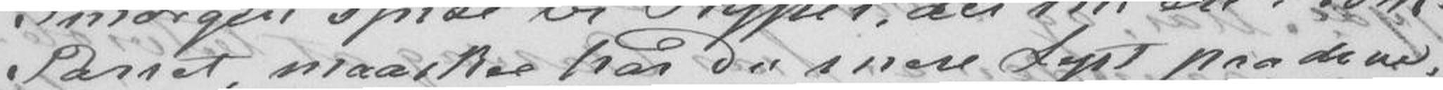Parret, maaskee har Du mere Lyst paa de nu,
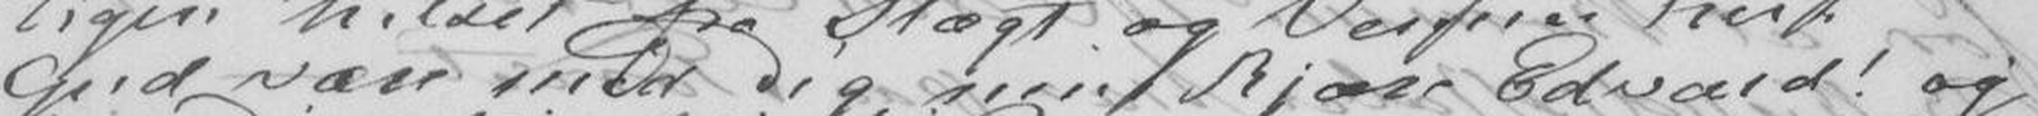Gud være med Dig, min kjære Edvard! og
